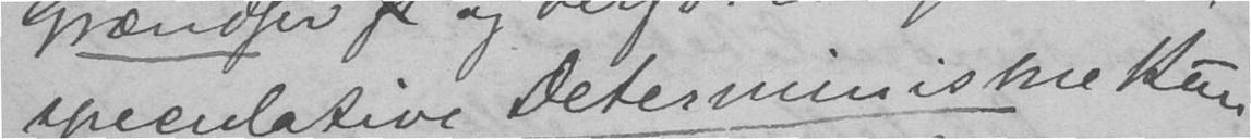speculative Determinisme kun
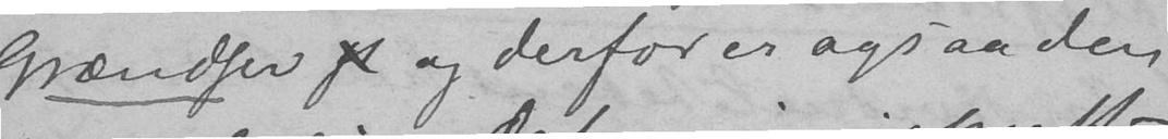Grændser og derfor er ogsaa den
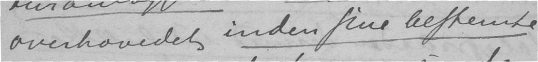overhovedet inden sine bestemte
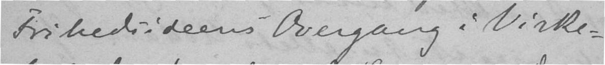Frihedsideens Overgang i Virke-
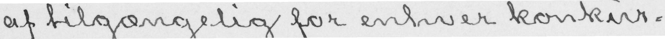af tilgængelig for enhver konkur-
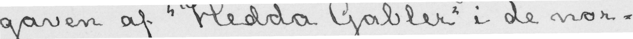gaven af «Hedda Gabler» i de nor-
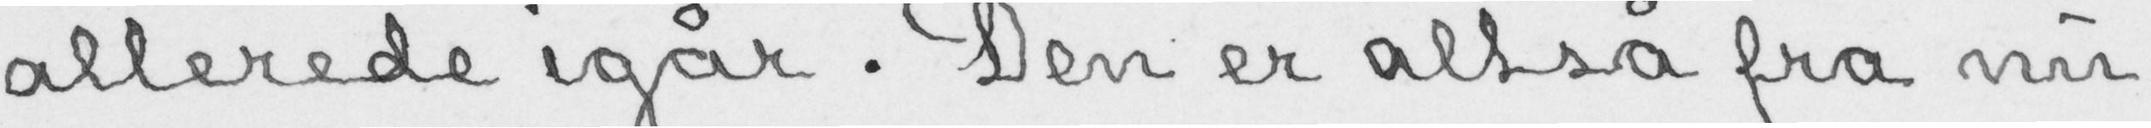allerede igår. Den er altså fra nu
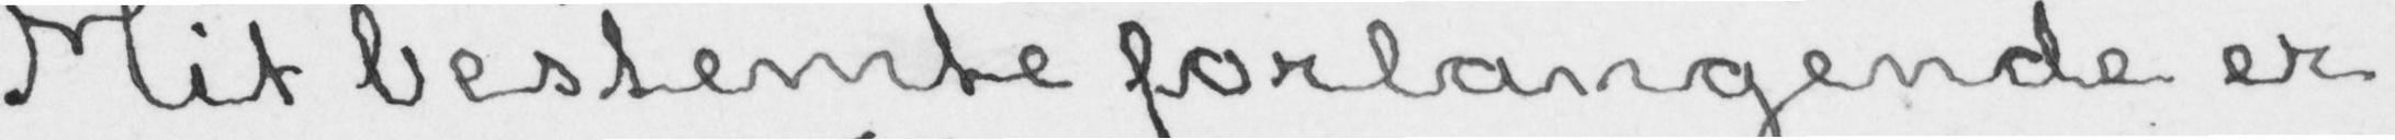Mit bestemte forlangende er
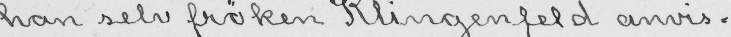han selv frøken Klingenfeld anvis-
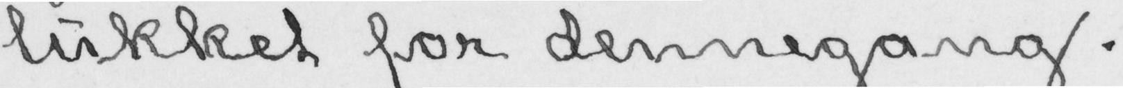lukket for dennegang.
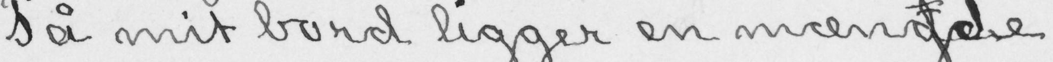På mit bord ligger en mændegde
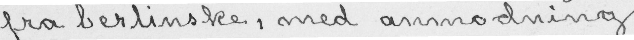fra berlinske, med anmodning
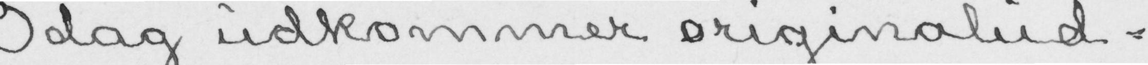Idag udkommer originalud-
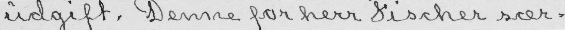udgift. Denne for herr Fischer sær-
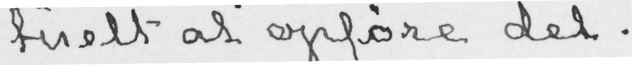tuelt at opføre det.
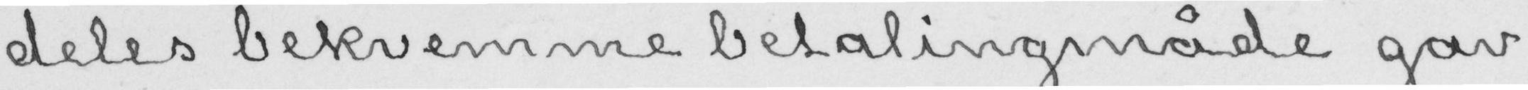deles bekvemme betalingsmåde gav
